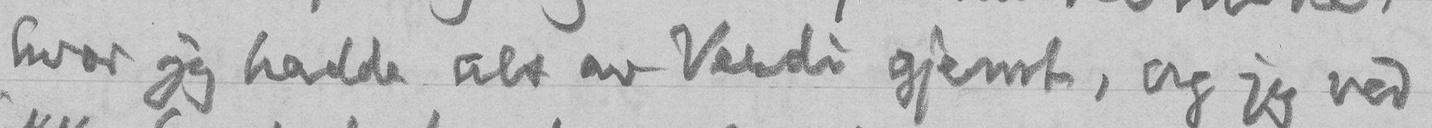hvor jeg hadde alt av Verdi gjemt, og jeg ved
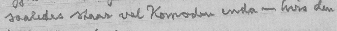saaledes staar vel Komoden enda - hvis den
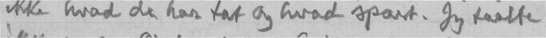ikke hvad de har tat og hvad spart. Jeg taalte
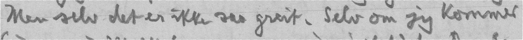Men selv det er ikke saa greit. Selv om jeg kommer
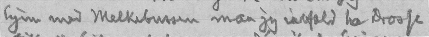hjem med Melkebussen maa jeg ialfald ha Drosje
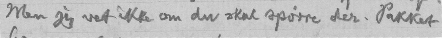Men jeg vet ikke om du skal spørre der. Pakket
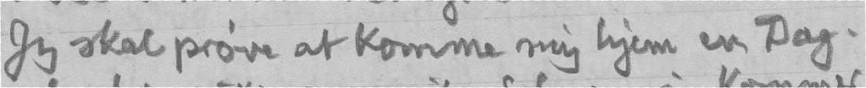Jeg skal prøve at komme mig hjem en Dag.
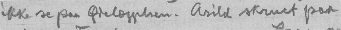ikke se paa Ødelæggelsen. Arild skruet paa
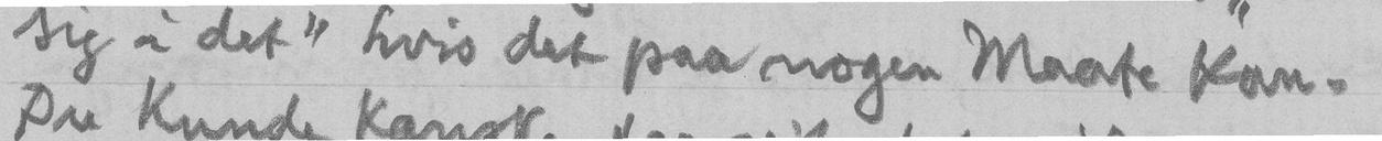sig i det" hvis det paa nogen Maate kan.
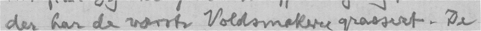der har de værste Voldsmakere grassert. De
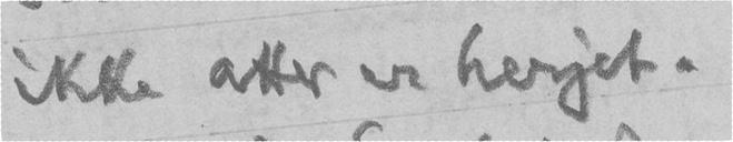ikke atter er herjet.
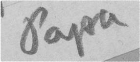Papa
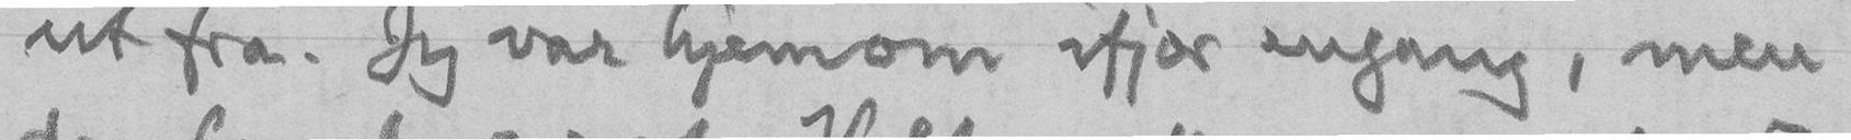ut fra. Jeg var hjemom ifjor engang, men
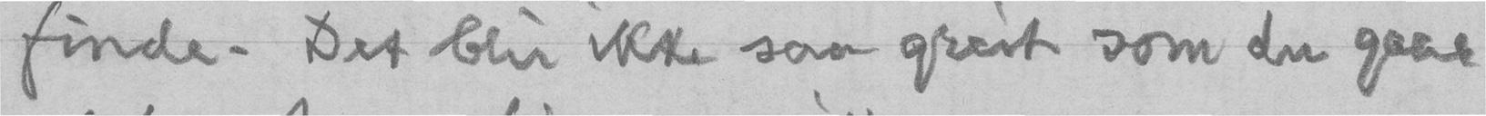finde. Det blir ikke saa greit som du gaar
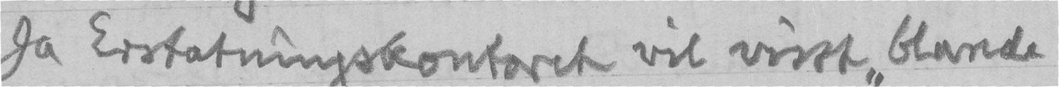Ja Erstatningskontoret vil visst "blande
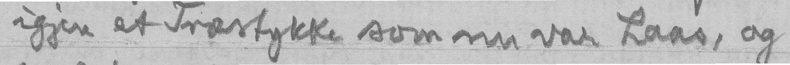igjen et Træstykke som nu var Laas, og
